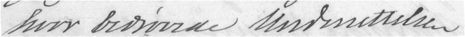hvor bedrøvede Underrettelsen
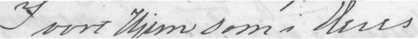I vore Hjem som i Deres
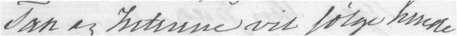Tak og Interesse vil følge hende
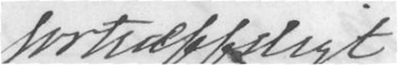fortræffeligt.
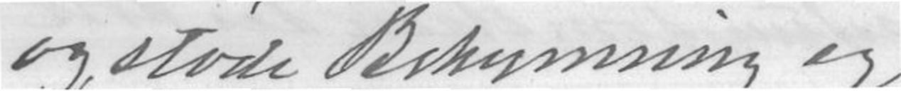- og, støde Bekymring og
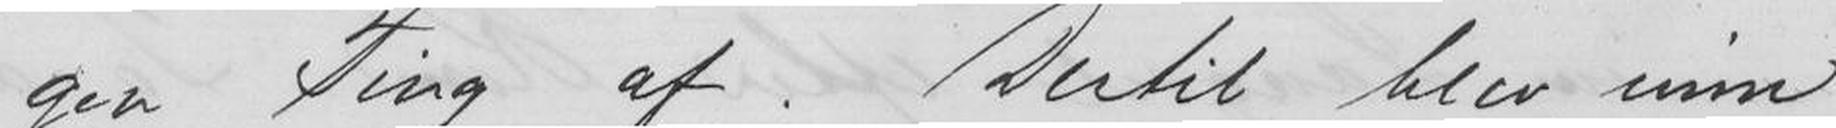gen Ting af. Dertil blev min
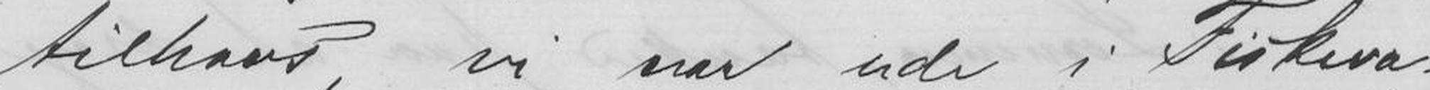tilhavs, vi var ude i Fiskeva-
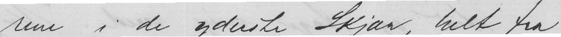rene i de yderste Skjær, helt fra
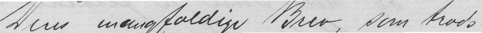Deres mangfoldige Brev, som trods
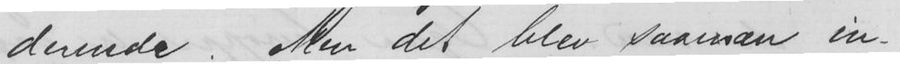dernede. Men det blev saamæn in-
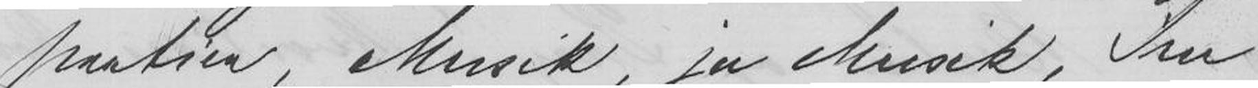partier, Musik, ja Musik, Fru
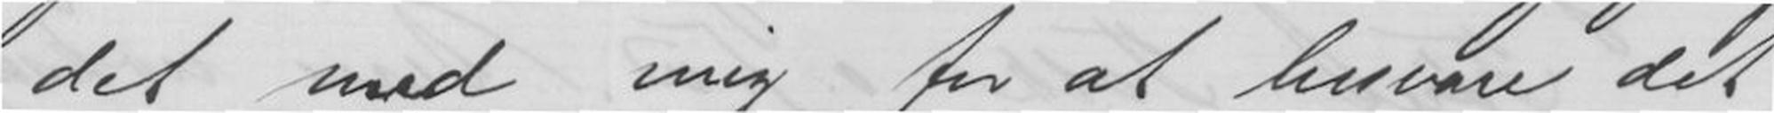det med mig for at besvare det
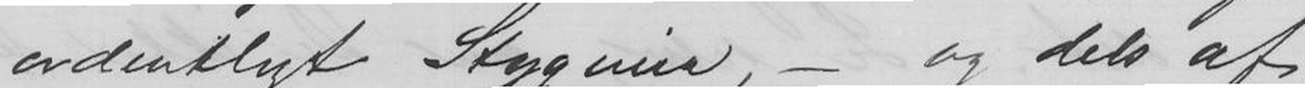ordentligt Stygveir, - og dels af
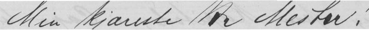Min kjæreste Hr Mester!
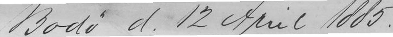Bodø d. 12 April 1885.
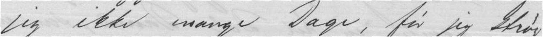jeg ikke mange Dage, før jeg støg
